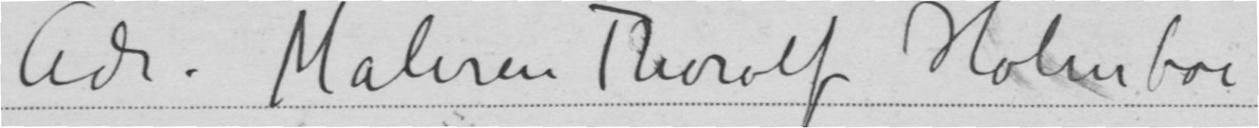Adr. Maleren Thorolf Holmboe
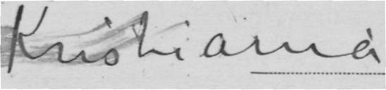Kristiania
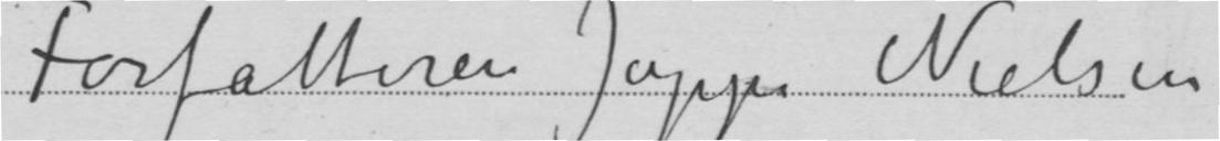Forfatteren Jappe Nielsen
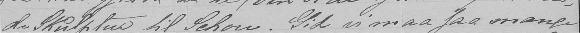de Skulptur til Schou. Gid vi maa faa mange
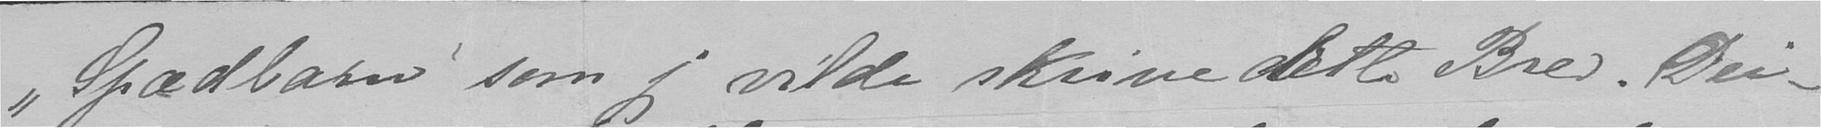"Spædbarn" som jeg vilde skrive dette Brev. Dei-
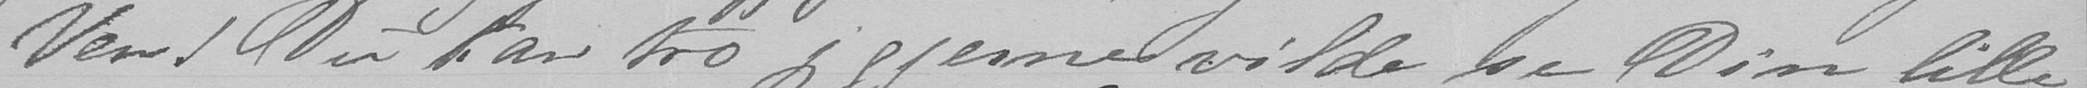Ven! Du kan tro jeg gjerne vilde se Din lille
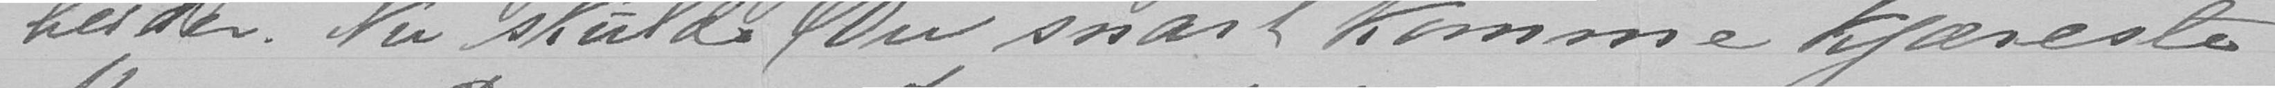beider. Nu skulde Du snart komme kjæreste
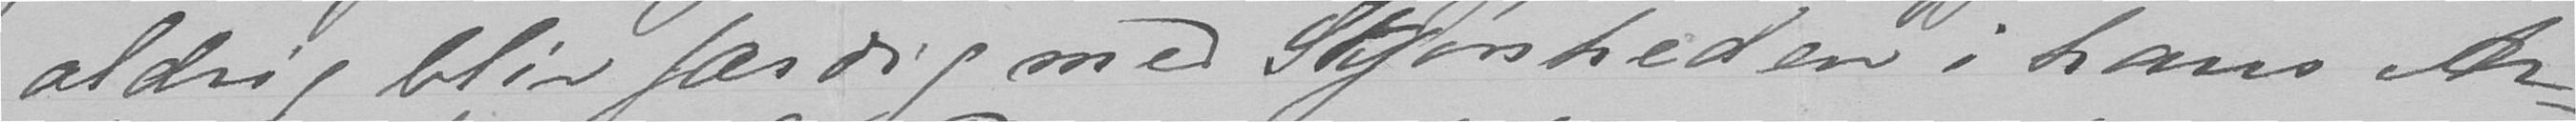aldrig blir færdig med Skjønheden i hans Ar-
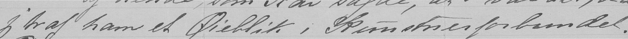jeg traf ham et Øieblik i Kunstnerforbundet.
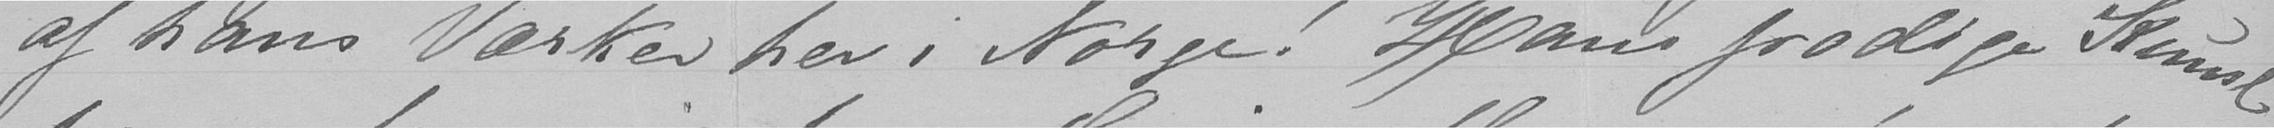af hans Værker her i Norge! Hans frodige Kunst
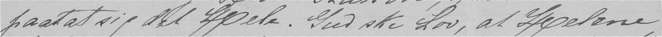paatat sig det Hele. Gud ske Lov, at Helene
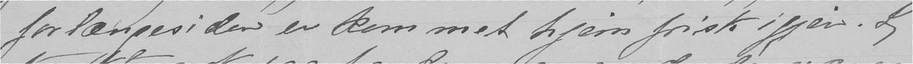for længesiden er kommet hjem frisk igjen. Jeg
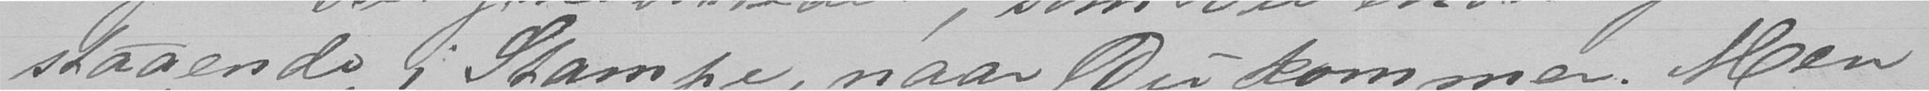staaende i Stampe, naar Du kommer. Men
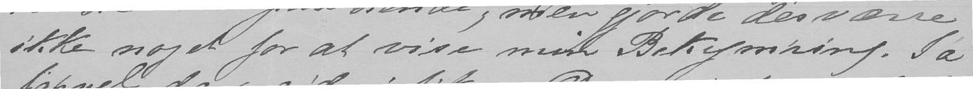ikke noget for at vise min Bekymring. Ja
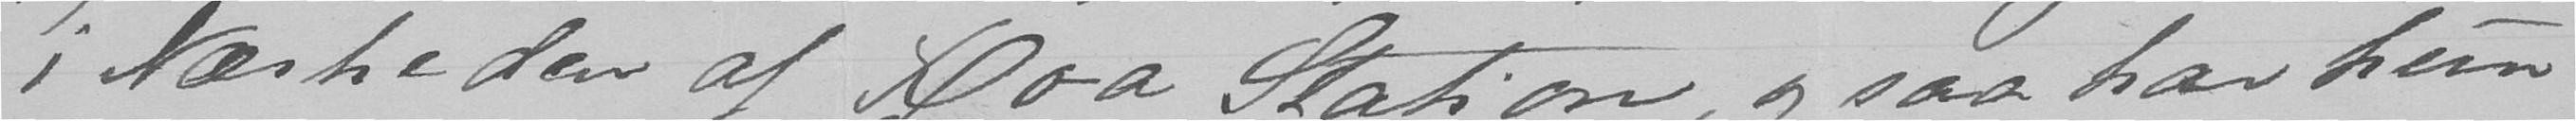i Nærheden af Roa Station, og saa har hun
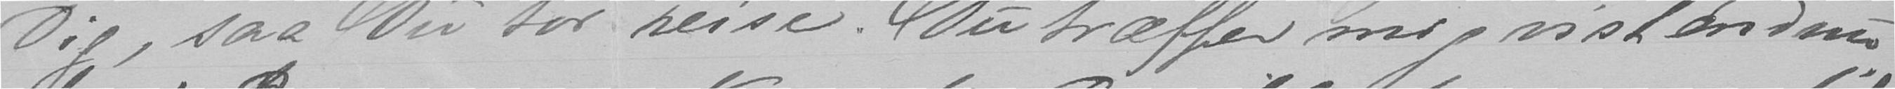Dig, saa Du tør reise. Du træffer mig vist endnu
